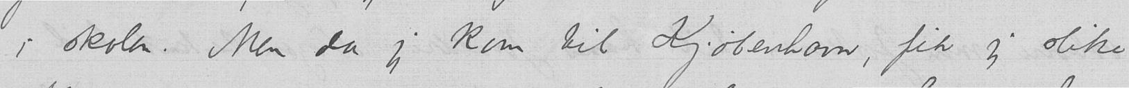i skolen. Men da jeg kom til Kjøbenhavn, fik jeg slike
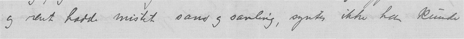og rent hadde mistet sans og samling, syntes ikke han kunde
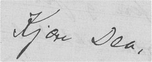Kjære Dea,
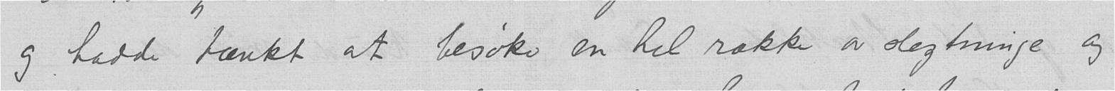og hadde tænkt at besøke en hel række av slegtninge og
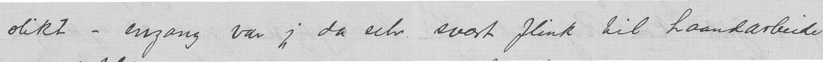slikt - engang var jeg da selv svært flink til haandarbeide
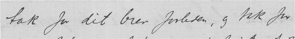tak for dit brev forleden, og tak for
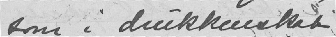som i drukkenskab
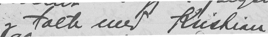og talte med Kristian
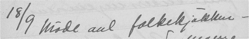18/9 Møde anl folkekjøkken -
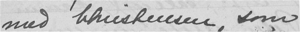med Christensen, som
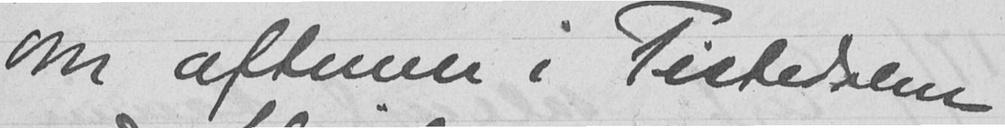Om aftenen i Tistedalen
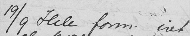19/9 Hele form. viet
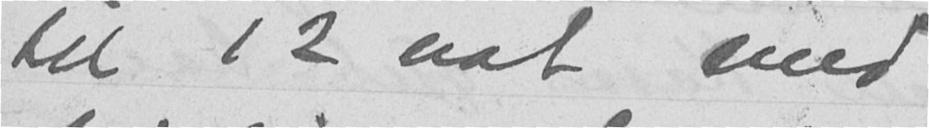til 12 nat med
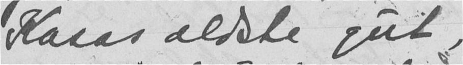Kasas ældste gut,
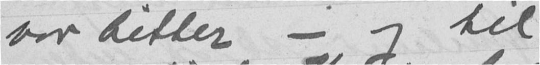var bitter - og til
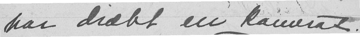har dræbt en kamerat.
